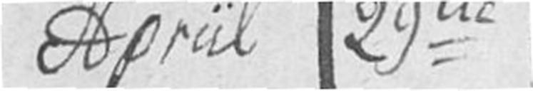Apriil 29de
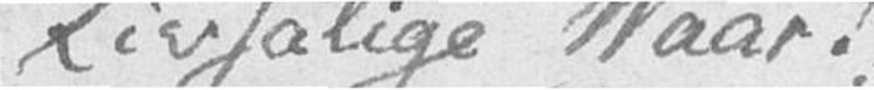Livsalige Vaar!
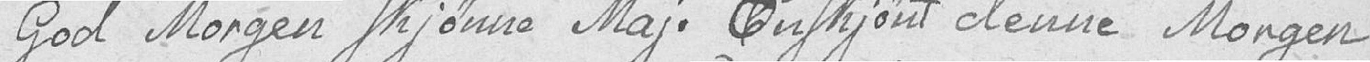God Morgen skjønne Maj! Enskjønt denne Morgen
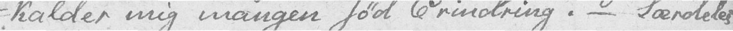-kalder mig mangen sød Erindring. – Særdeles
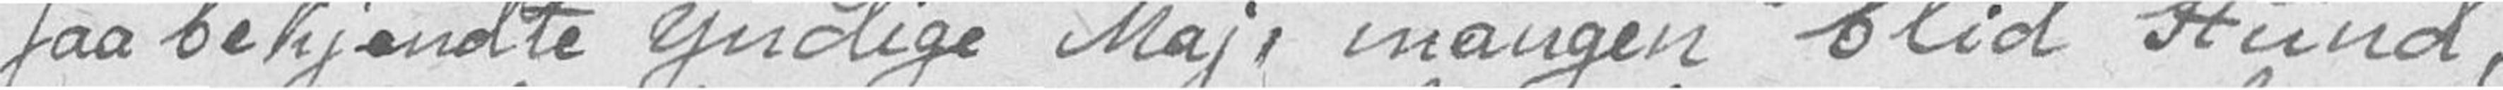saa bekjendte yndige Maj, mangen blid Stund,
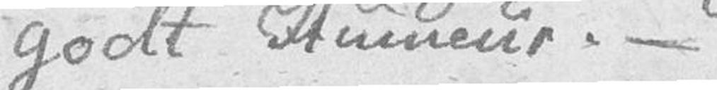godt Humeur. –
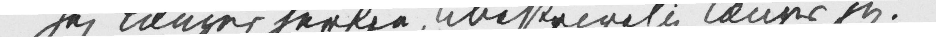Jeg længes herfra, ubeskrivelig længes jeg,
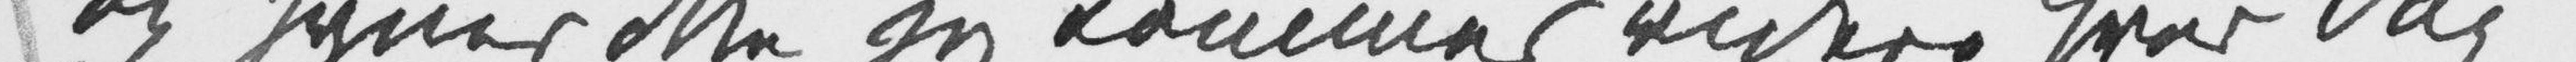og synes ikke jeg kommer videre hver Dag
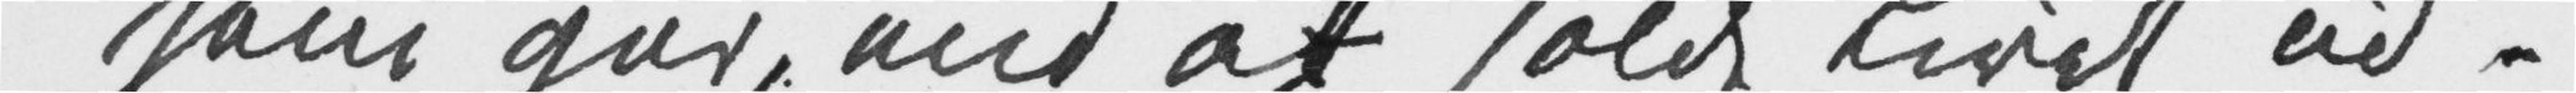som går, end at holde Livet ud.
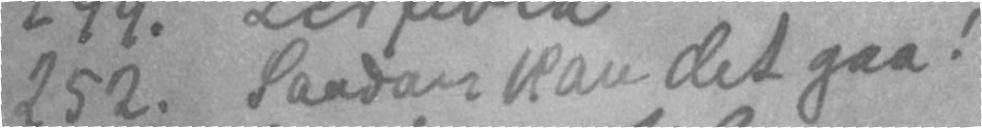252. Saadan kan det gaa!
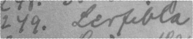249. Lerfibla
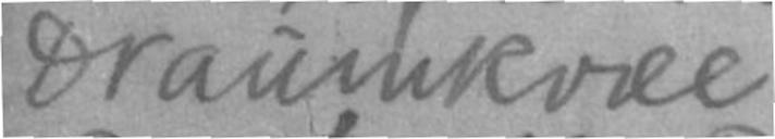Draumkvæe
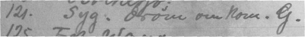121. Syg. drøm om kom. G.
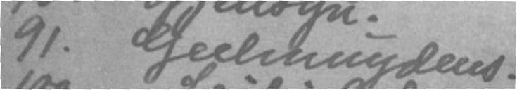91. Geelmuydens.
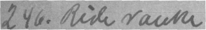246. Ride ranke
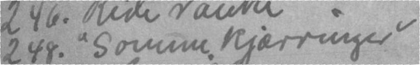248. "Somme kjærringer"
